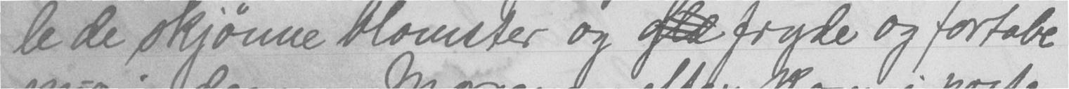le de skjønne blomster og glæ fryde og fortabe
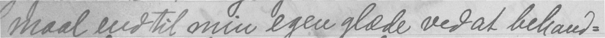maal end til min egen glæde ved at behand-
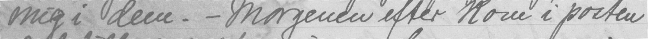mig i dem. - Morgenen efter kom i posten
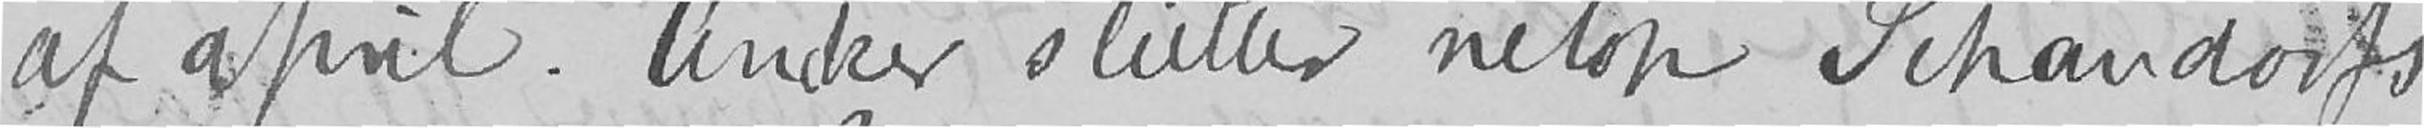af april. Ancker slutter netop Schandorfs
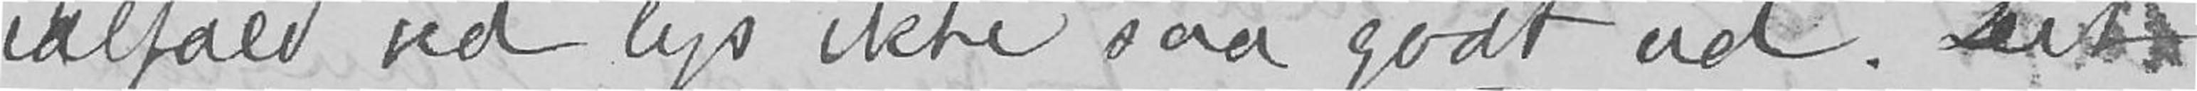ialfald ved lys ikke saa godt ud. Det
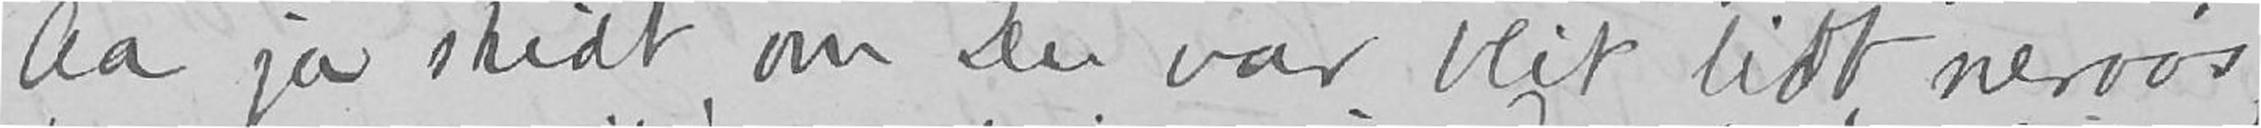Aa ja skidt om Du var blit lidt nervøs
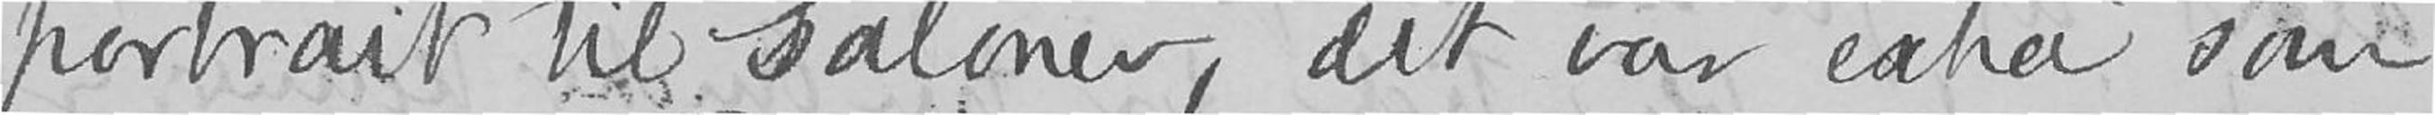portrait til Salonen, det var extra som
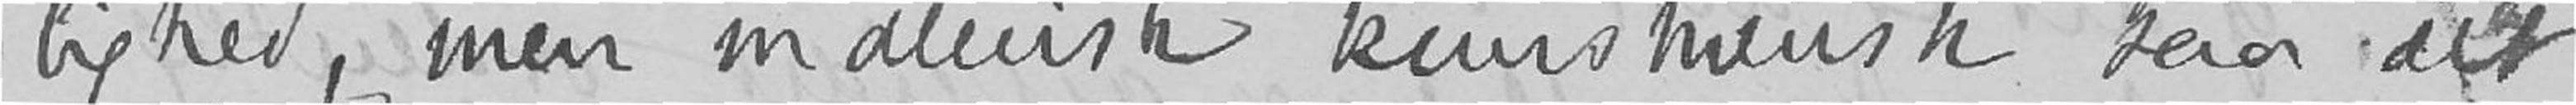lighed, men malerisk kunstnerisk saa det
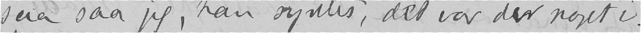saa saa jeg, han syntes, det var der noget i.
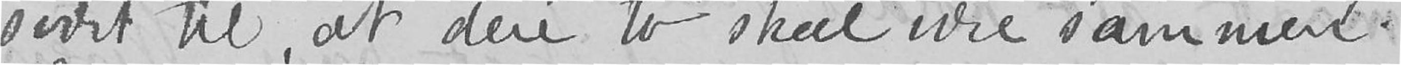svært til, at dere to skal være sammen.
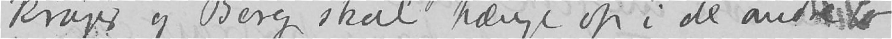Krøyer og Berg skal hænge op i de andre to
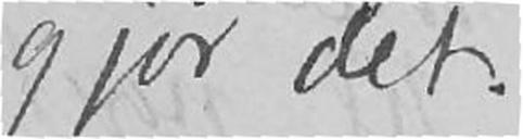gjør det.
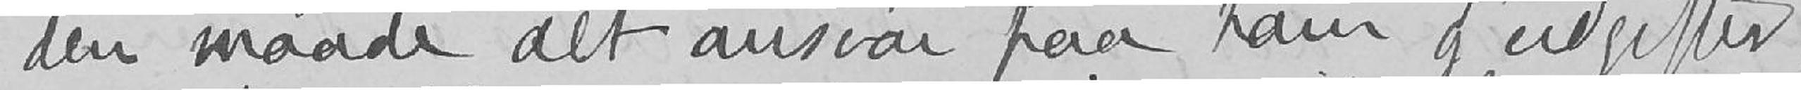den maade alt ansvar paa ham og udgifter,
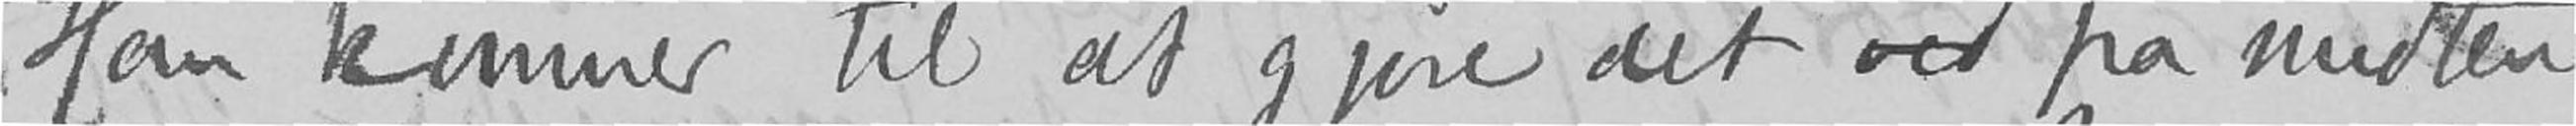Han kommer til at gjøre det ved fra midten
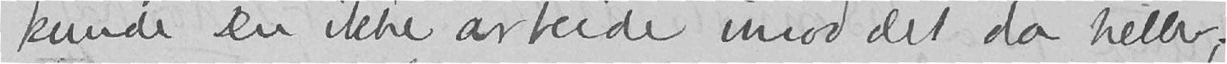kunde Du ikke arbeide imod det da heller;»
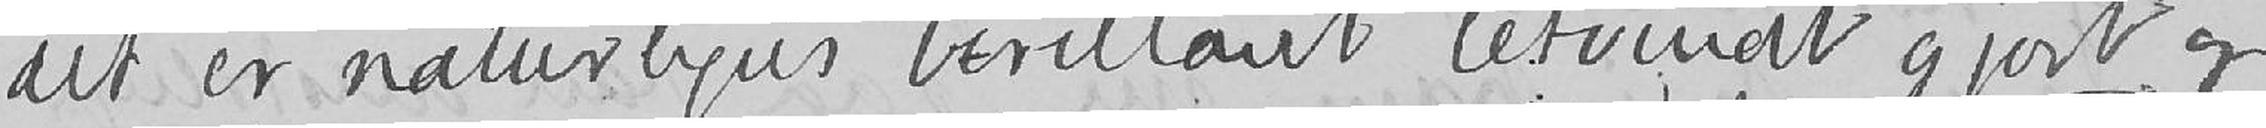det er naturligvis berillant letvindt gjort og
